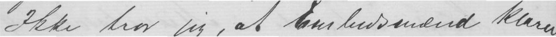Ikke tror jeg, at Embedsmænd klarer
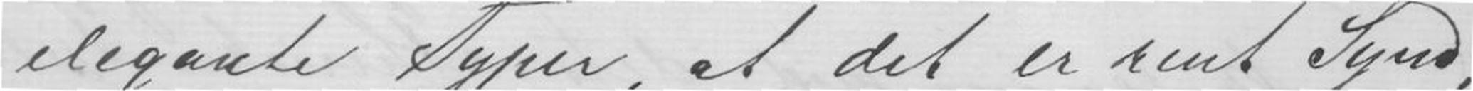elegante Typer, at det er rent Synd,
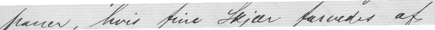paner, hvis fine Skjær farvedes af
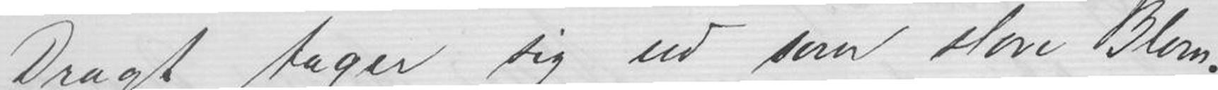Dragt tager sig ud som store Blom-
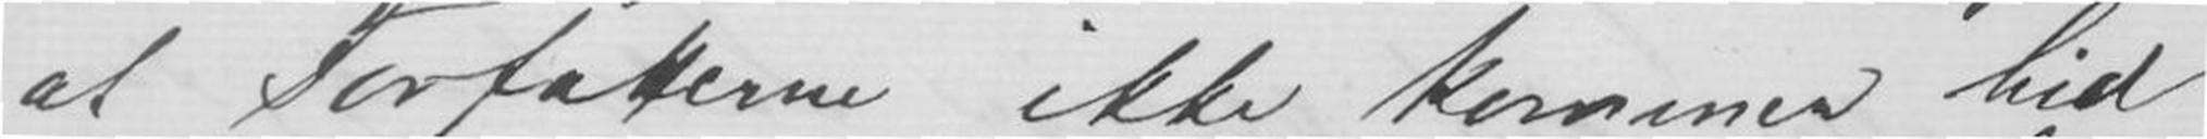at Forfatterne ikke kommer hid
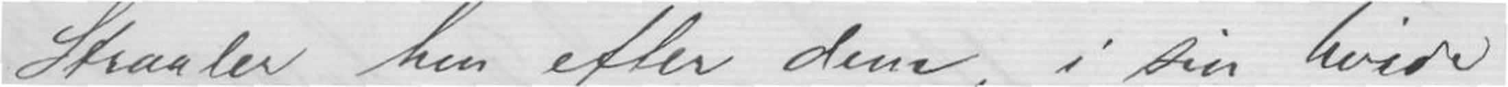Straaler hen efter dem, i sin hvide
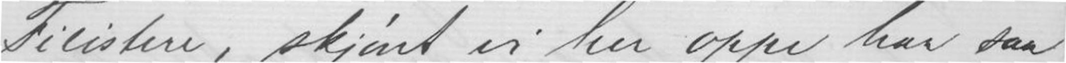Filistere, skjønt vi her oppe har saa
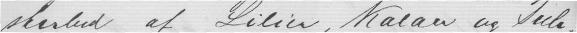sterbed af Lilier, Kalaer og Tule-
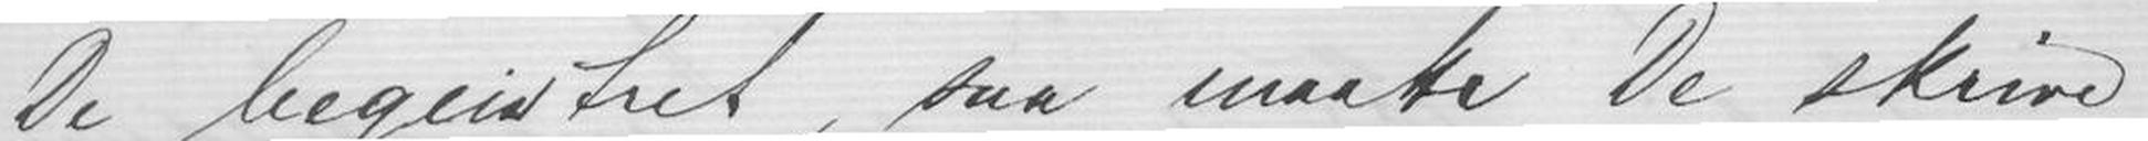De begeistret, saa maatte De skrive
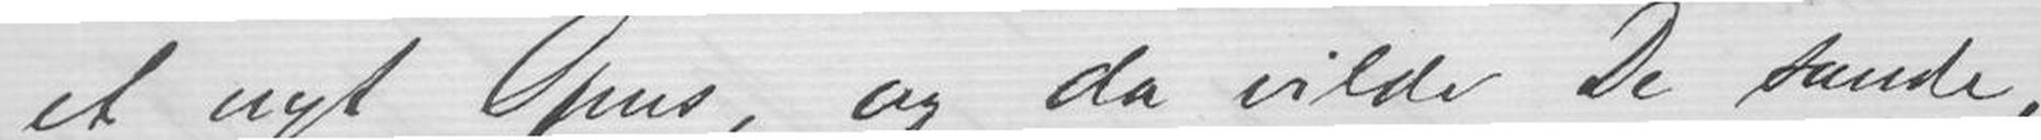et nyt Opus, og da vilde De sande,
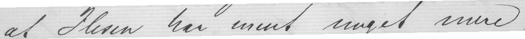at Ibsen har ment meget mere.
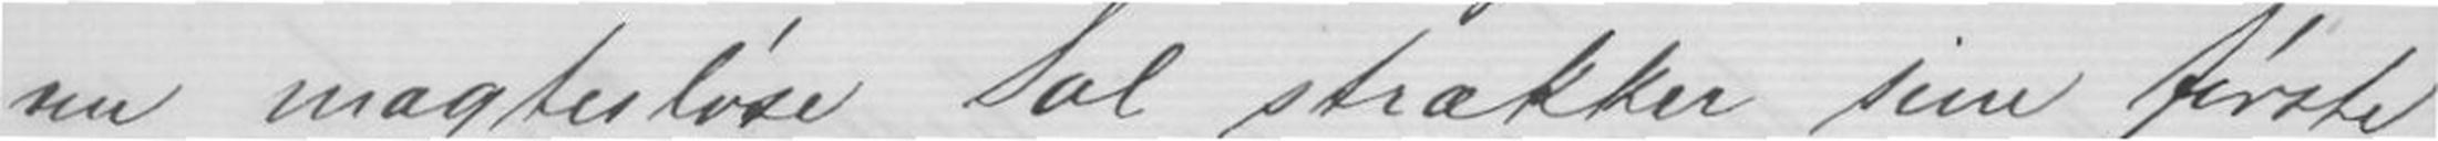nu magtesløse Sol strækker sine første
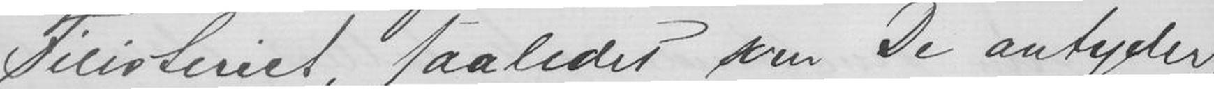Filisteriet, saaledes som De antyder.
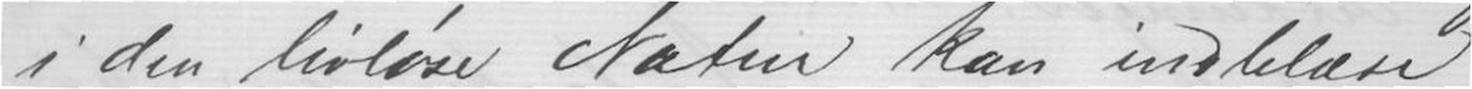i den livløse Natur kan indblæse
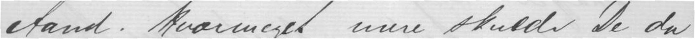Aand. hvormeget mere skulde De da
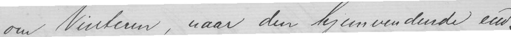om Vinteren, naar den hjemvendende end-
